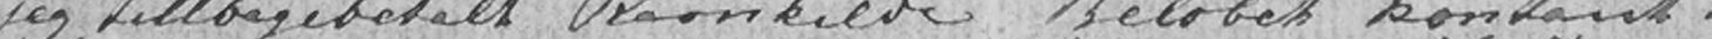har jeg tillbagebetalt Ravnkilde Beløbet kontant.
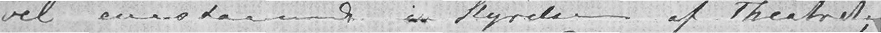vel enestaaende i Styrelsen af Theatret;
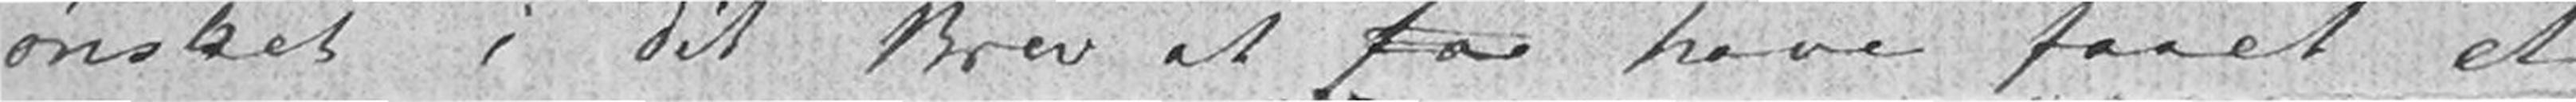ønsket i dit Brev at have faaet et
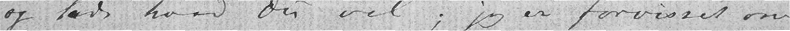og lade hvad Du vil; jeg er forvisset om
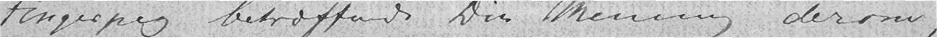Fingerpeg betræffende Din Mening derom;
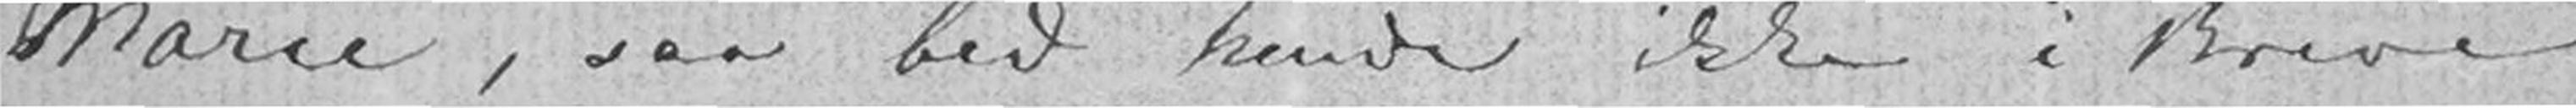Marie, saa bed hende ikke i Breve
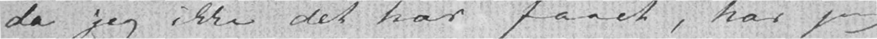da jeg ikke det har faaet, har jeg
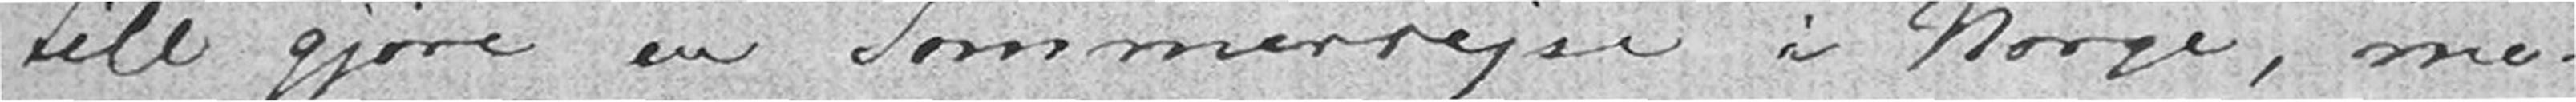till gjøre en Sommerrejse i Norge, me-
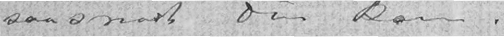saasnart Du kan.
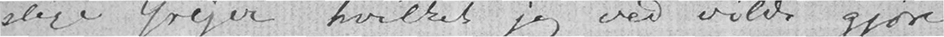slige Grejer hvilket jeg ved vilde gjøre
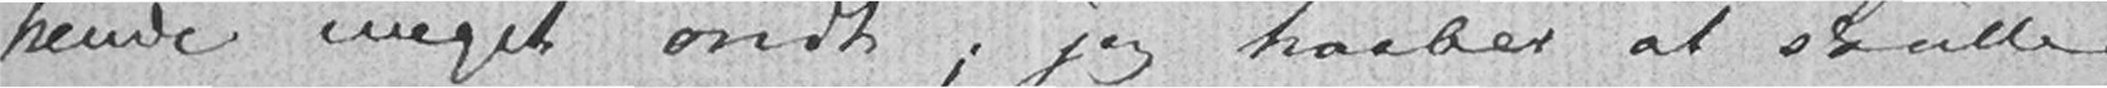hende meget ondt; jeg haaber at skulle
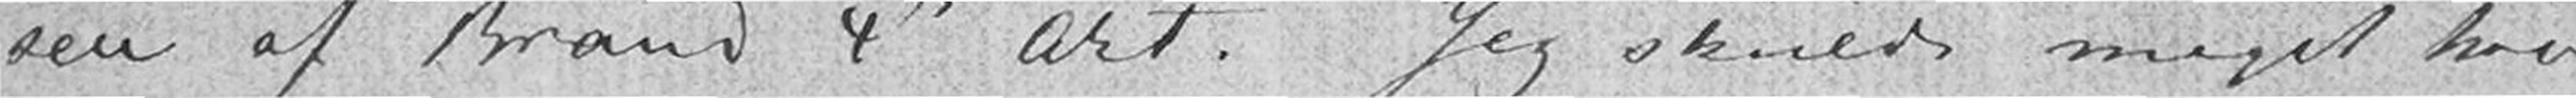sen af Brand 4de Akt. Jeg skulde meget have
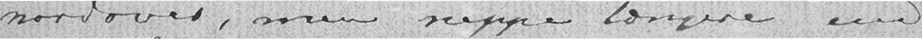nordover, men neppe længere end
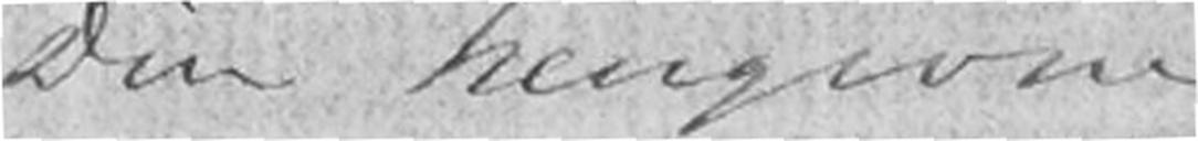Din hengivne
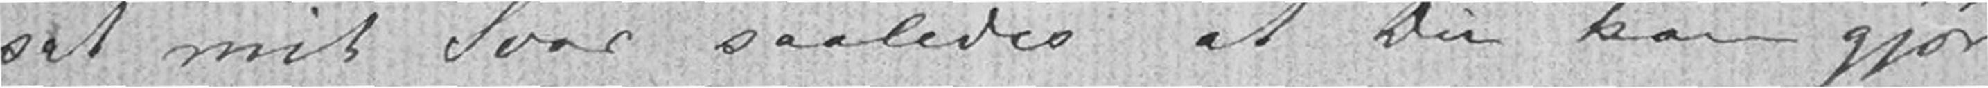sat mit Svar saaledes at Du kan gjøre
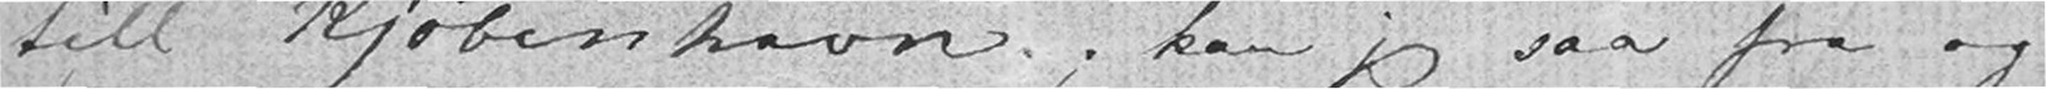till Kjøbenhavn; kan jeg saa fra og
